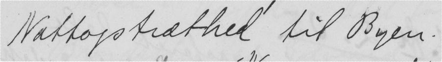Nattogstræthed til Byen.
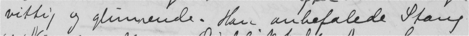vittig og glimrende. Han anbefalede Stang
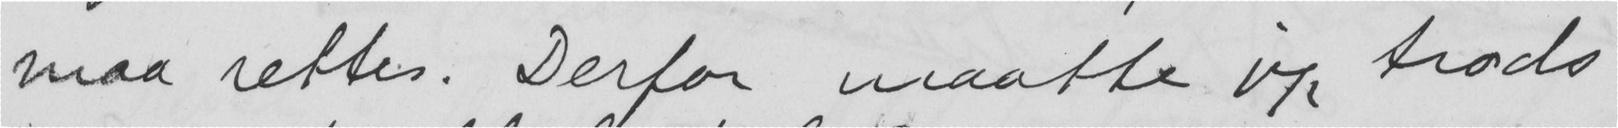maa rettes. Derfor maatte jeg trods
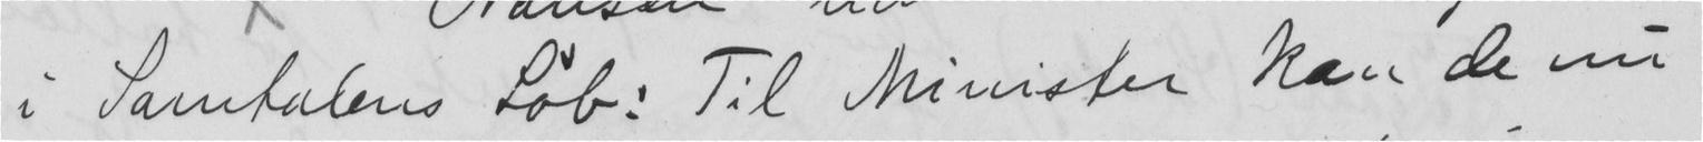i Samtalens Løb: Til Minister kan de nu
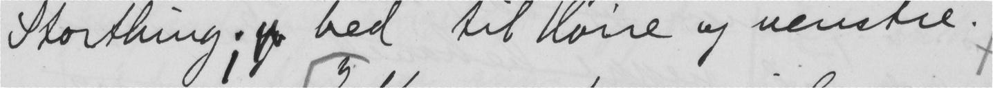Storthing;  bed til Høire og venstre.
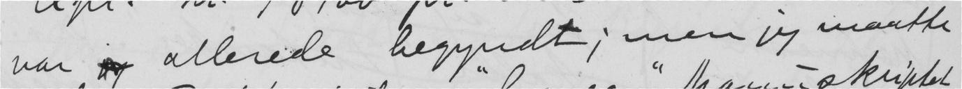var  allerede begyndt; men jeg maatte
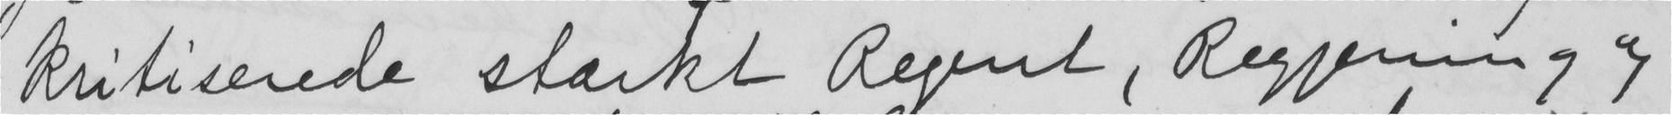Kritiserede stærkt Regent, Regjening og
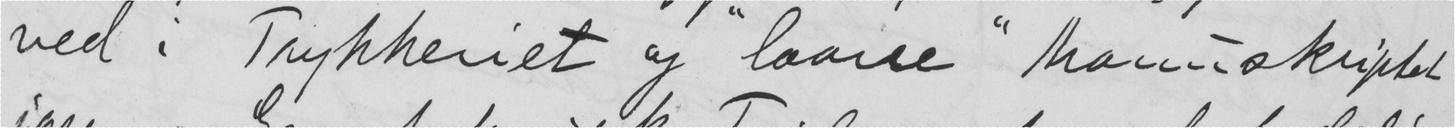ned i Trykkeret og "laane" Manuskriptet
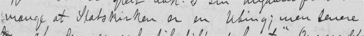mange at Statskirken er en Uting; men senere
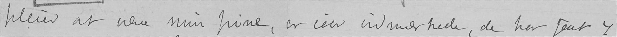pleier at være min pine, er iaar udmærkede, de har faat 7
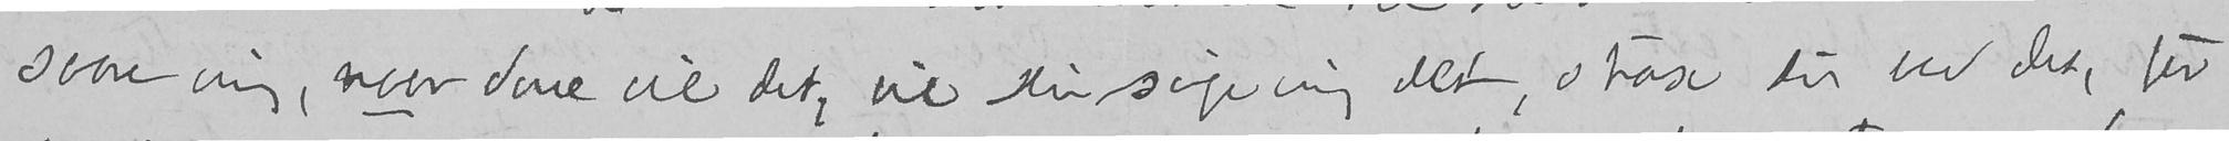svare mig, naar dere vil det, vil Du sige mig det, strax du ved det, for
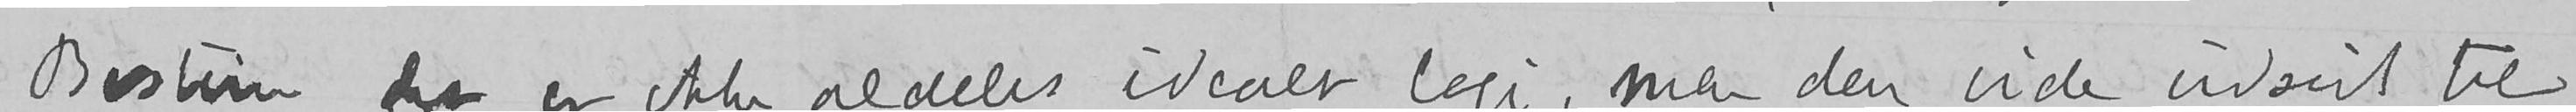Bestum, det er ikke aldeles idealt logi, men den vide udsigt til
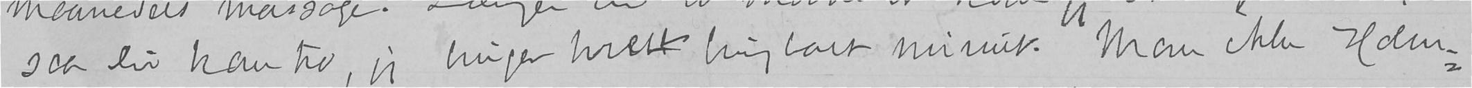saa du kan tro, jeg bruger hvert brugbart minut. Mon ikke Holm-
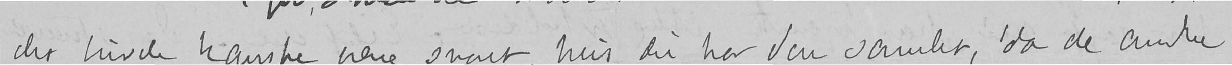det burde kanske være snart, hvis du har den samlet, da de andre
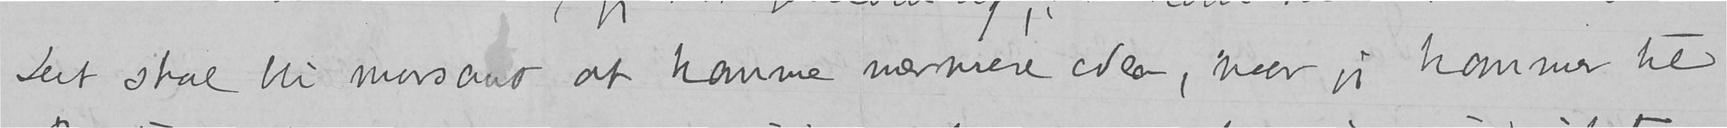Det skal bli morsomt at komme nærmere eder, naar jeg kommer til
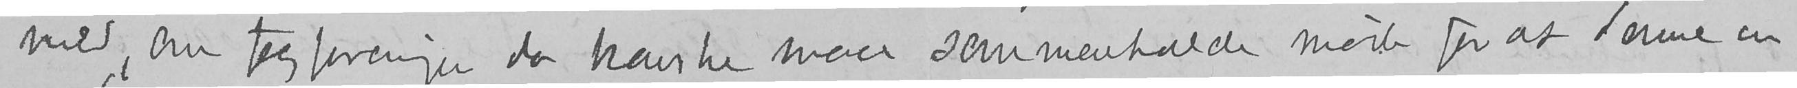med, om fagforeningen da kanske maa sammenholde møde for at danne en
